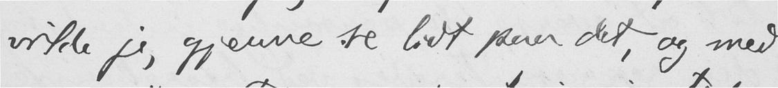vilde jeg gjerne se lidt paa det, og med
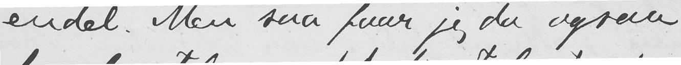endel. Men saa faar jeg da ogsaa
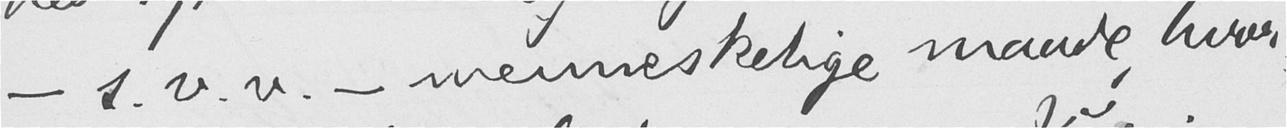- s. v. v. - menneskelige maade, hvor-
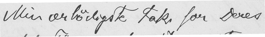Min ærbødigste tak for Deres
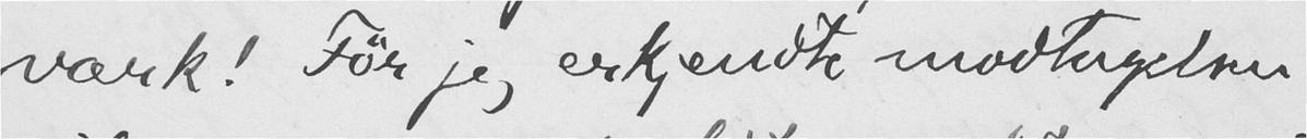værk! Før jeg erkjendte modtagelsen
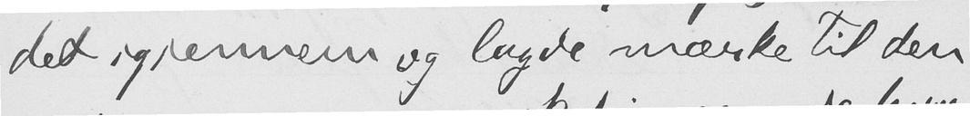det igjennem og lagde mærke til den
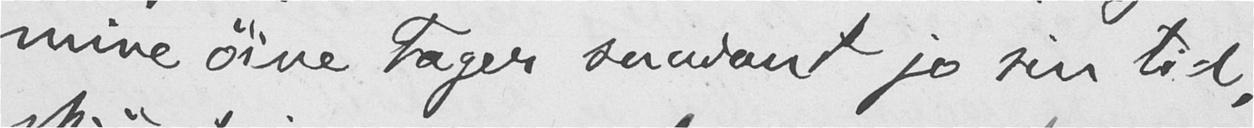mine øine tager saadant jo sin tid,
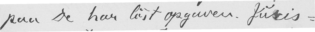paa De har løst opgaven. Juris-
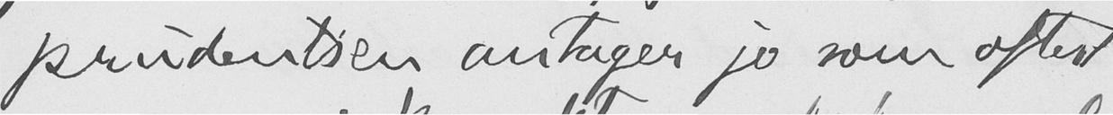prudentsen antager jo som oftest
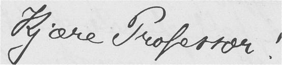Kjære Professor!
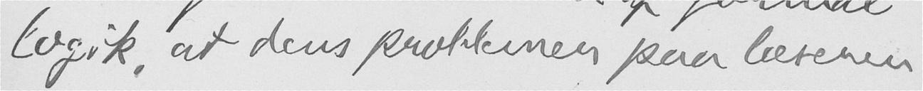logik, at dens problemer paa læseren
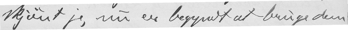skjønt jeg nu er begyndt at bruge dem
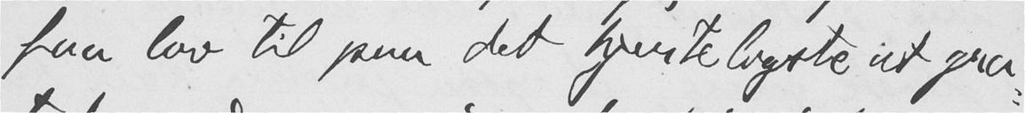faa lov til paa det hjerteligste at gra-
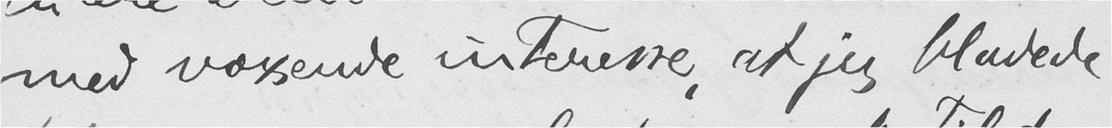med voxende interesse, at jeg bladede
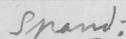Spand.
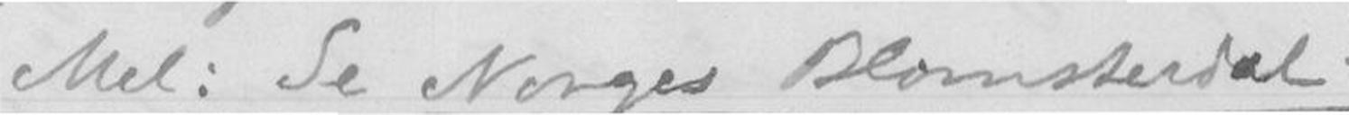Mel: Se Norges Blomsterdal
